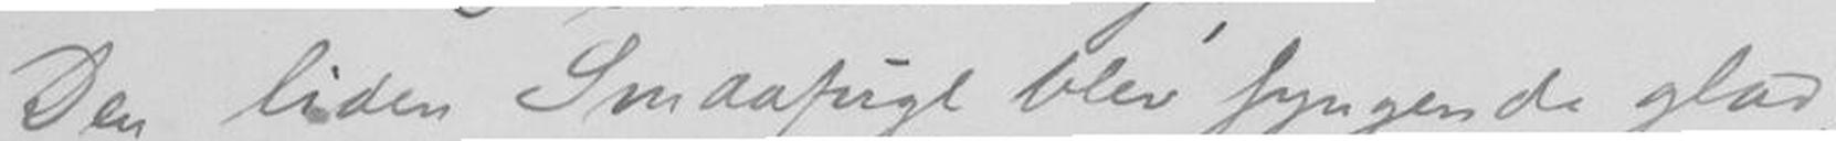Den liden Smaafugl blev syngende glad,
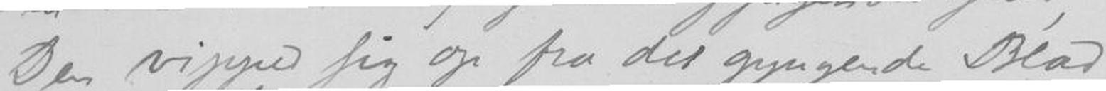Den vipped sig op fra det gyngende Blad
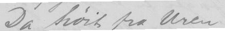Da høit fra Uren
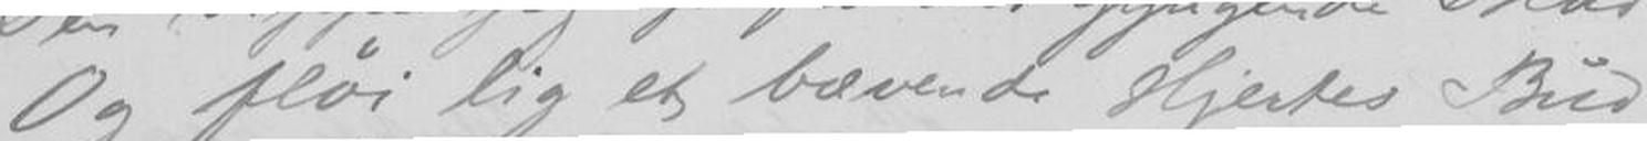Og fløi lig et bævende Hjertes Bud
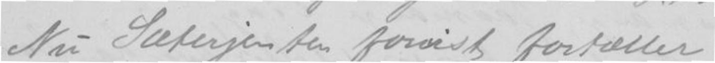Nu Sæterjenten forvist fortæller
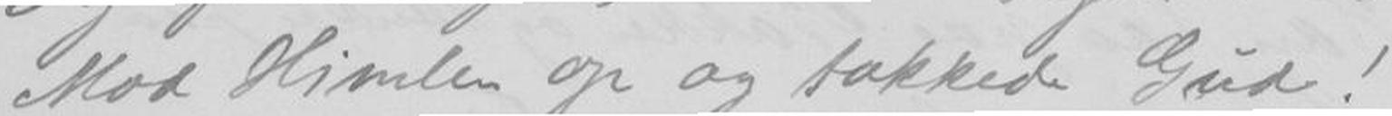Mod Himlen op og takket Gud!
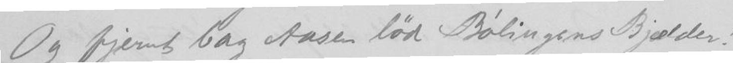Og fjernt bag Aasen lød Bølingens Bjælder!
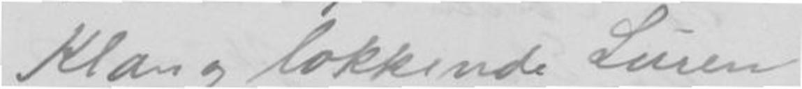Klang lokkende Luren
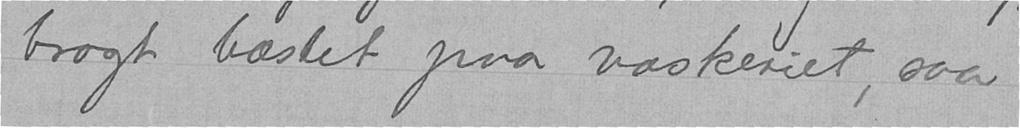bragt bæstet paa vaskeriet, saa
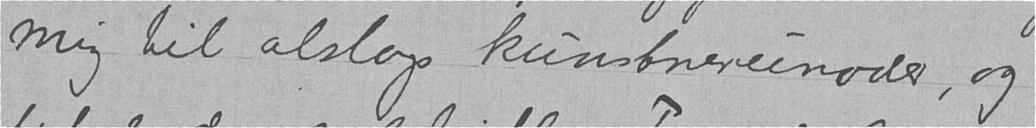mig til alslags kunstnerunoder, og
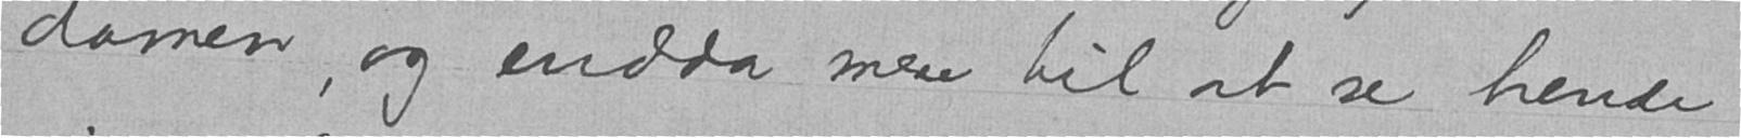damen, og endda mere til at se hende
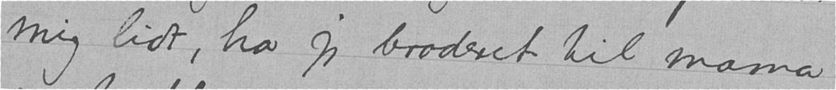mig lidt, har jeg broderet til mama
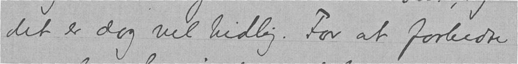det er dog vel tidlig. For at forbedre
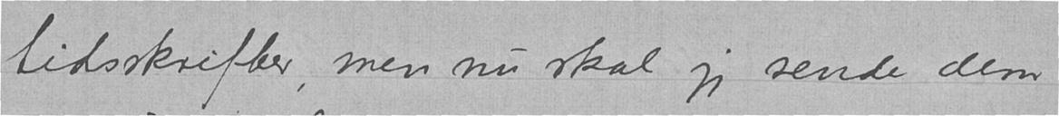tidsskrifter, men nu skal jeg sende dem
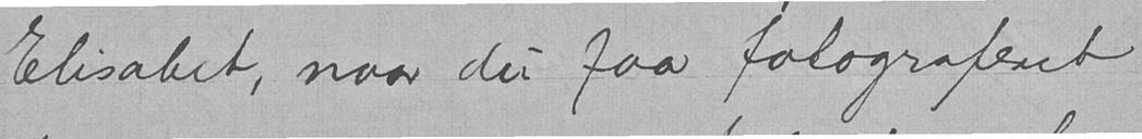Elisabet, naar du faar fotograferet
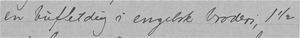en buffetdug i engelsk broderi, 1½
